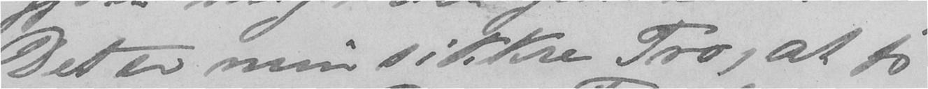Det er min sikkre Tro, at jeg
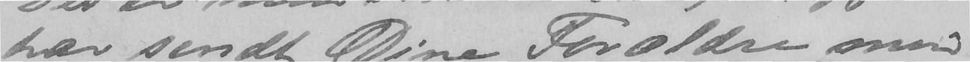har sendt Dine Forældre min
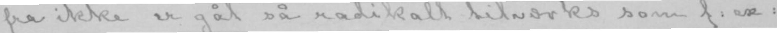fra ikke er gåt så radikalt tilværks som f: ex:
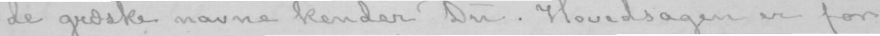de græske navne kender Du. Hovedsagen er for
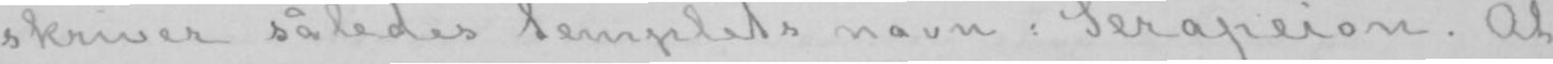skriver således templets navn: Serapeion. At
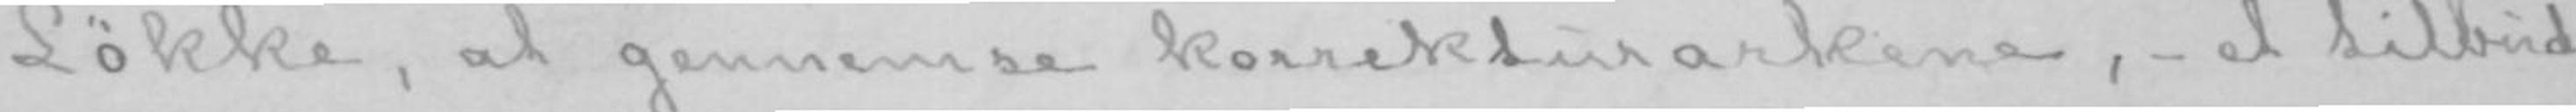Løkke, at gennemse korrekturarkene,- et tilbud,
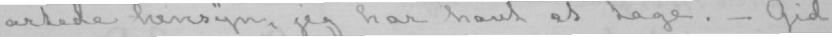artede hensyn, jeg har havt at tage.- Gid
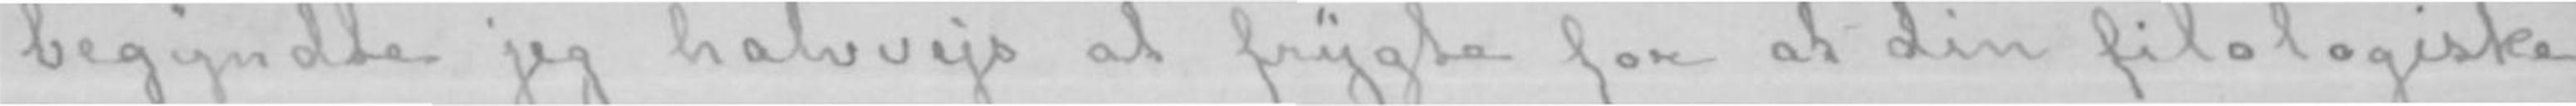begyndte jeg halvvejs at frygte for at din filologiske
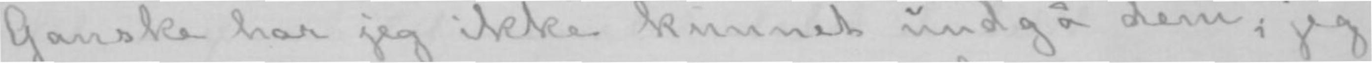Ganske har jeg ikke kunnet undgå dem; jeg
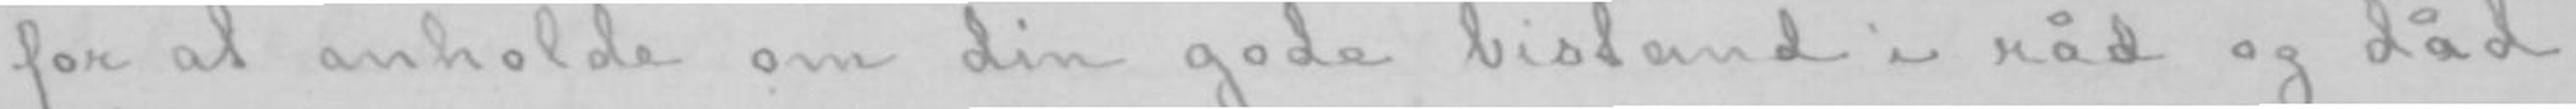for at anholde om din gode bistand i råd og dåd.
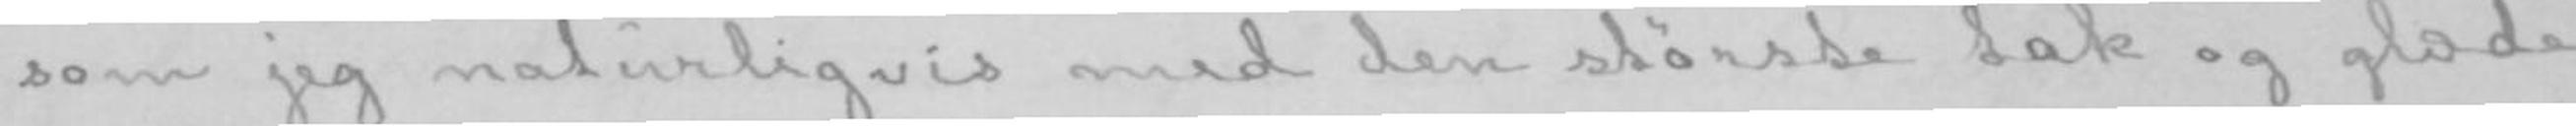som jeg naturligvis med den største tak og glæde
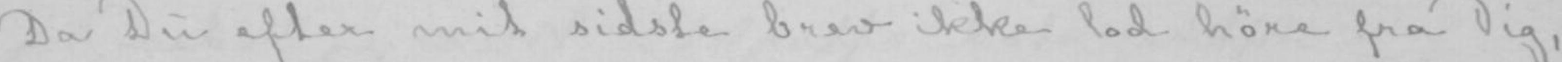Da Du efter mit sidste brev ikke lod høre fra Dig,
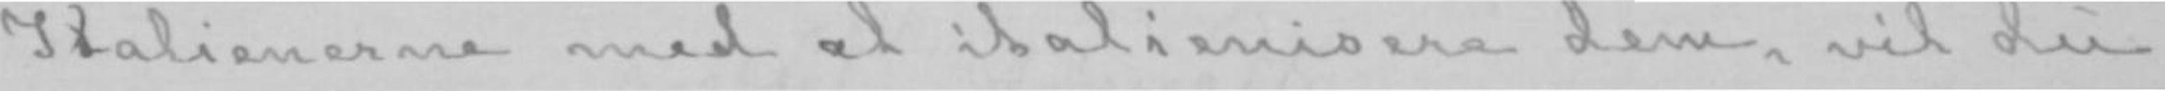Italienerne med at italienisere dem, vil du
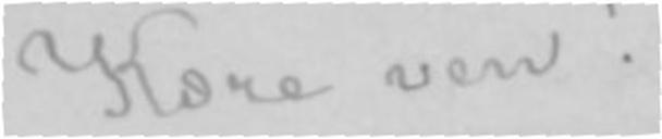Kære ven!
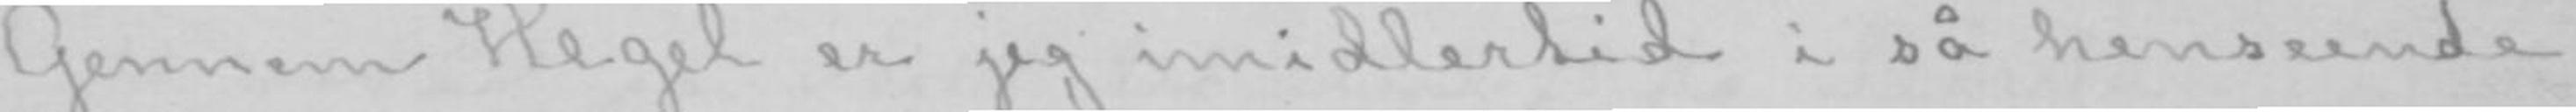Gennem Hegel er jeg imidlertid i så henseende
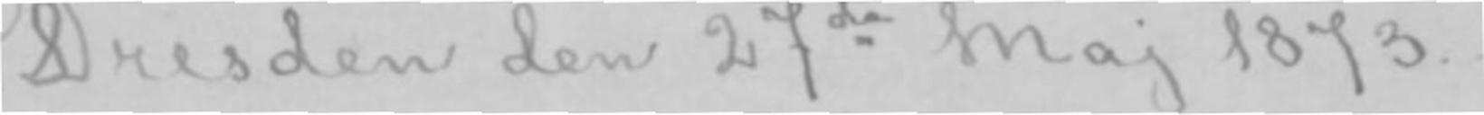Dresden den 27de Maj 1873.-
